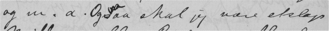og m. a. Ogsaa skal jeg være etslags
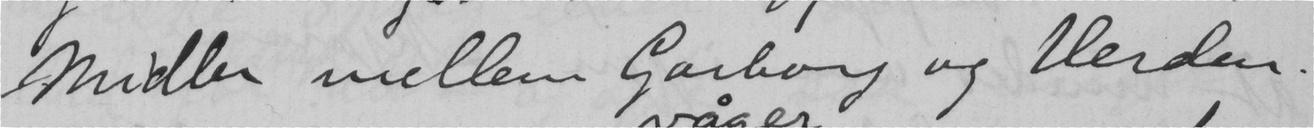midler mellem Garborg og Verden.
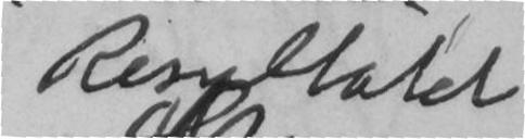Resultatet
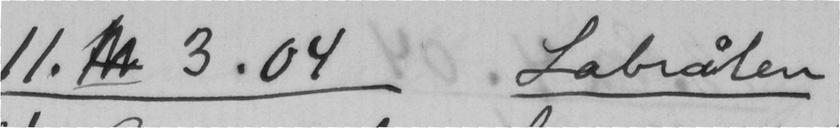11. 3.04 Labråten
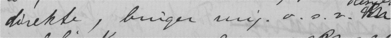direkte, bruger mig. o. s. v.
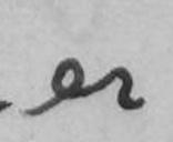er
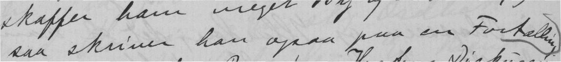saa skriver han ogsaa paa en Fortælling.
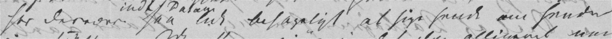har desværre indt Datum saa lidt behageligt at sige hende om hendes
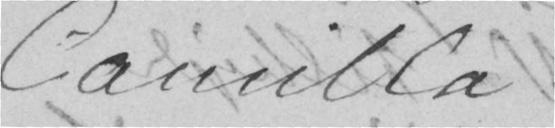Camilla
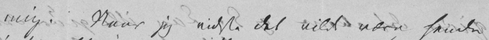mig. Naar jeg vidste det vilde være hende
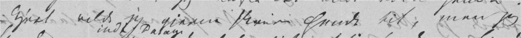kjert vilde jeg gjerne skrive hende til, men jeg
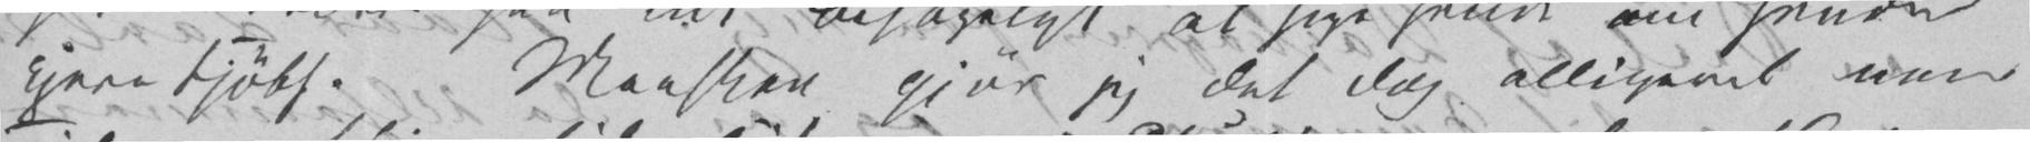kjere Kjøbh. Maaskee gjør jeg det dog alligevel naar
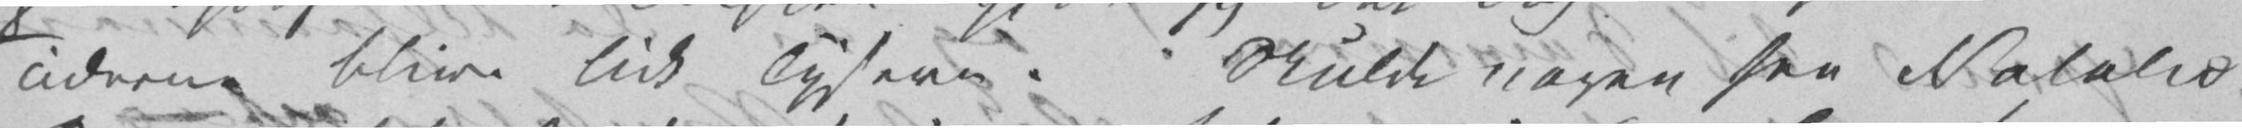Tiderne blive lidt lysere. Skulde nogen see Natalie
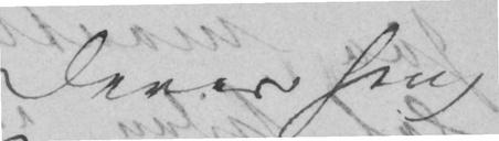Deres heng
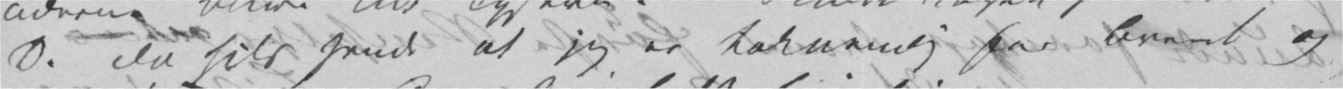D. da hils hende at jeg er taknemlig for Brevet og
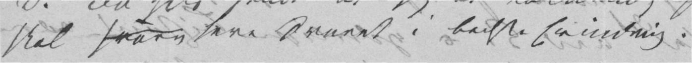skal svare have Svaret i bedste Erindring.
